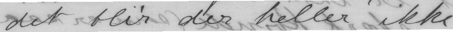det blir der heller ikke
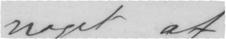noget af
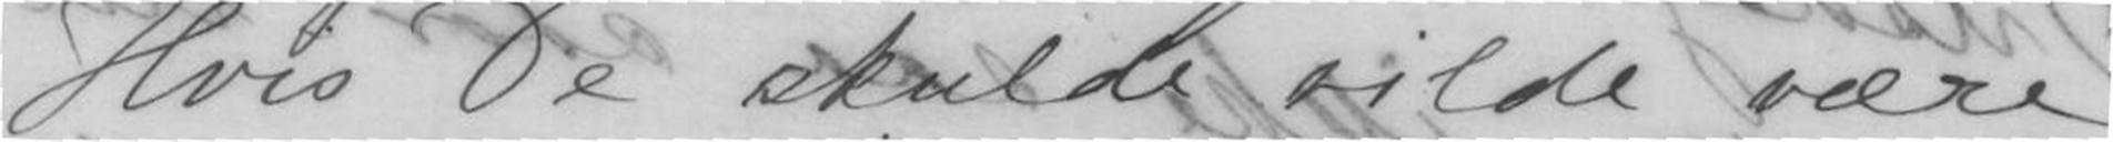Hvis De skulde vilde være
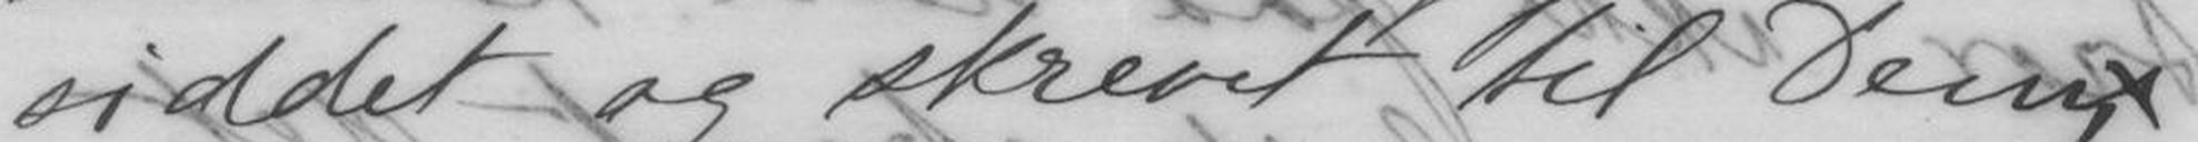siddet og skrevet til Dem
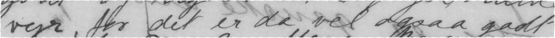vejr, for der er da vel ogsaa godt
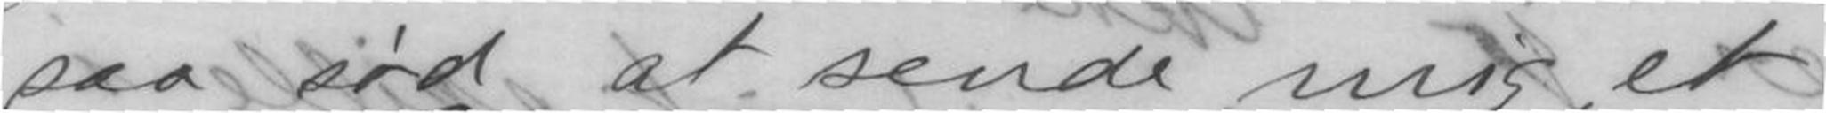saa sød at sende mig et
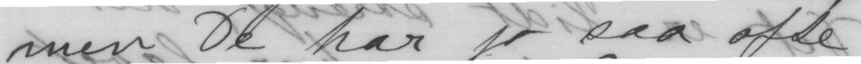men De har jo saa ofte
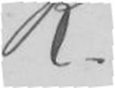R.
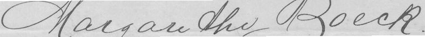Margarethe Boeck
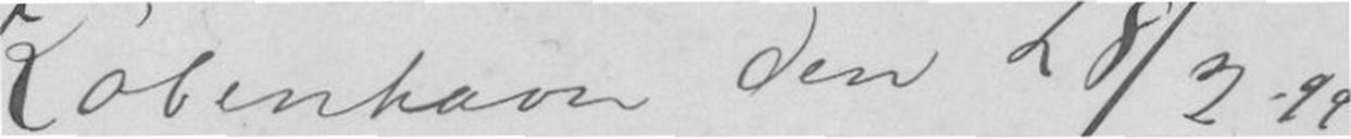København den 28/2. 99.
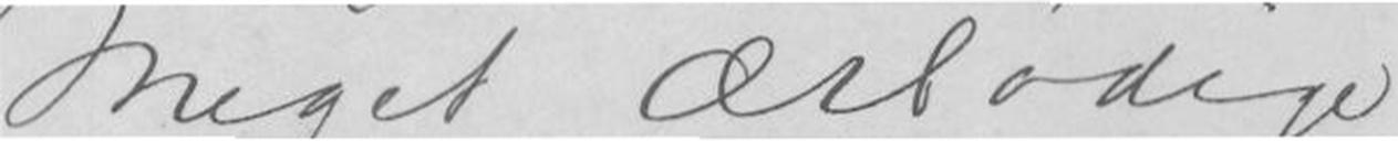Meget ærbødige
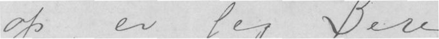op, er jeg Deres
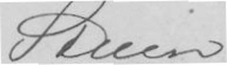Stuen
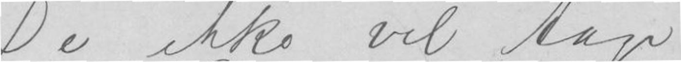De ikke vil tage
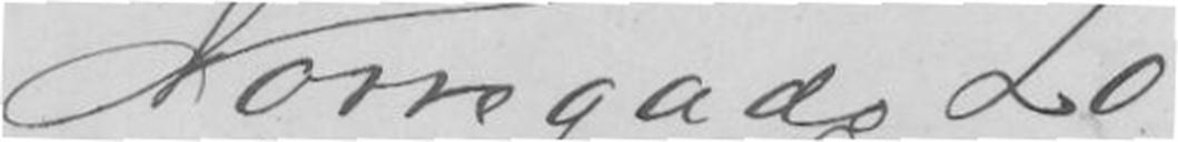Nørregade 20
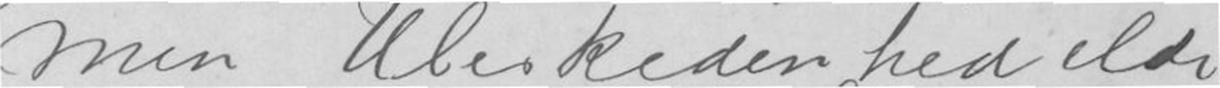min Ubeskedenhed ilde
